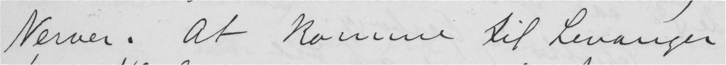Nerver. At komme til Levanger
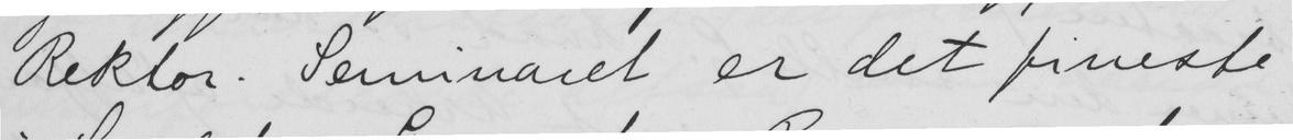Rektor. Seminaret er det fineste
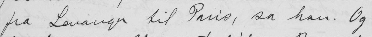fra Levanger til Paris, sa han. Og
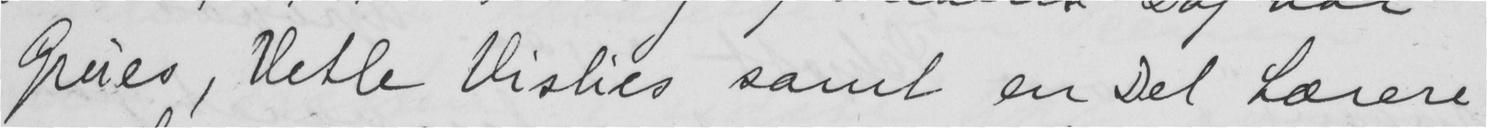Grues, Vetle Vislies samt en Del Lærere
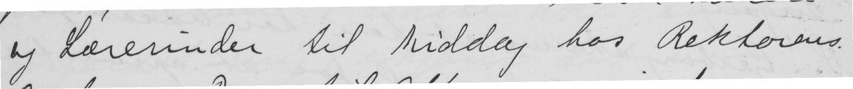og Lærerinder til Middag hos Rektorens.
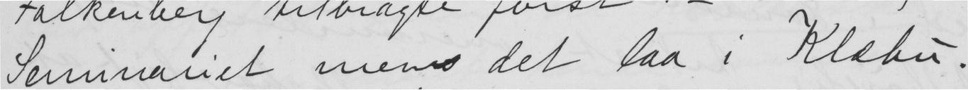Semiariet mens det laa i Klæbu.
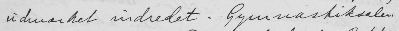udmærket indredet. Gymnastiksalen
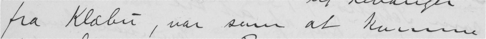fra Klæbu, var som at komme
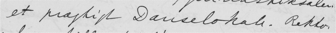et prægtigt Danselokale. Rektor
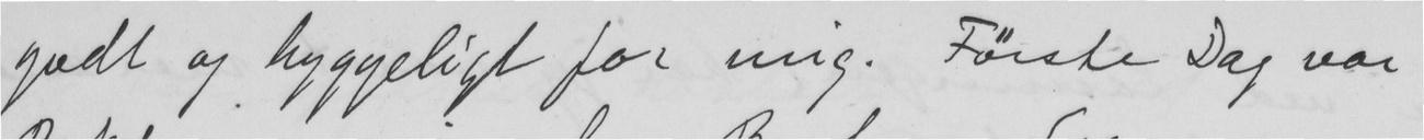godt og hyggeligt for mig. Første Dag var
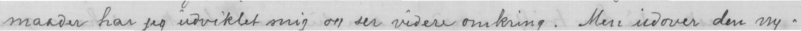maader har jeg udviklet mig og ser videre omkring. Men udover den ny-
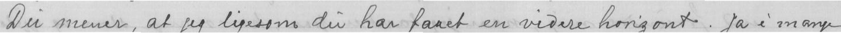Du mener, at jeg ligesom du har faaet en videre horisont. Ja i mange
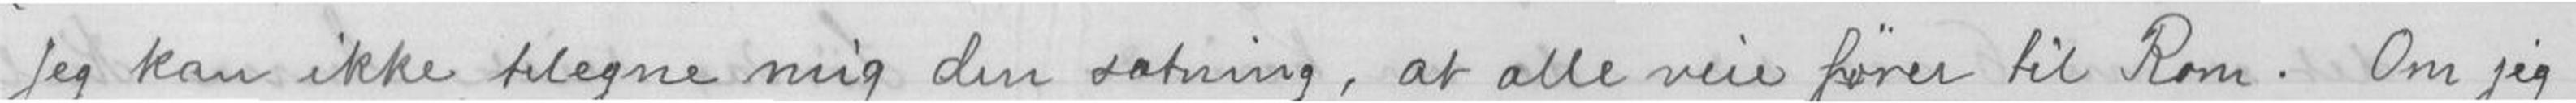Jeg kan ikke tilegne mig den sætning, at alle veie fører til Rom. Om jeg
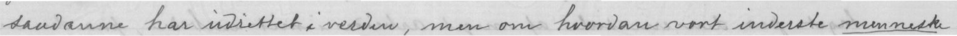saadanne har udrettet i verden, men om hvordan vort inderste menneske-
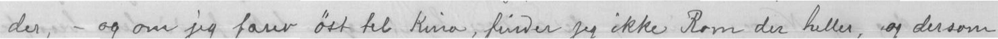der, - og om jeg farer øst til Kina, finder jeg ikke Rom der heller, og dersom
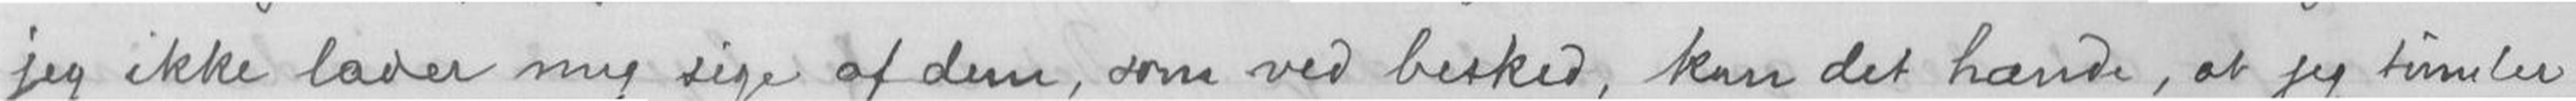jeg ikke lader mig sige ag dem, som ved besked, kan det hænde, at jeg tumler
